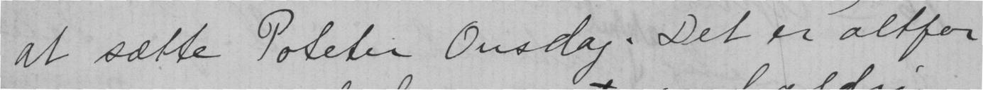at sætte Poteter Onsdag. Det er altfor
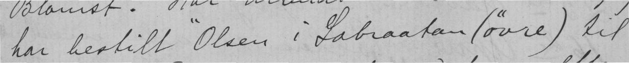har bestilt "Olsen i Lobraatan (øvre) til
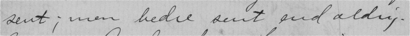sent; men bedre sent end aldrig.
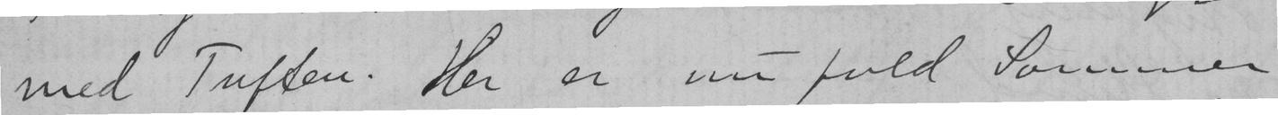med Tuften. Her er nu fuld Sommer
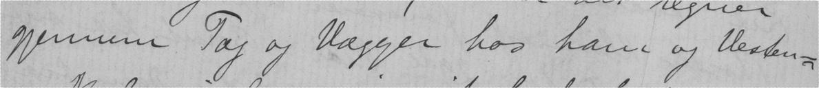gjennem Tag og Vægger hos ham og Vesten-
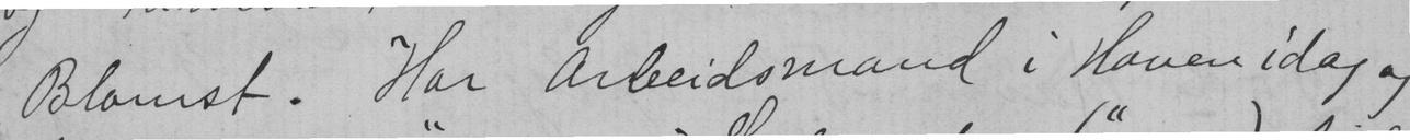Blomst. Har Arbeidsmand i Haven idag og
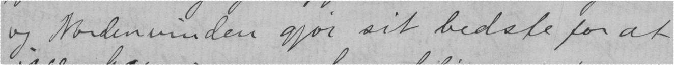og Nordenvinden gjør sit bedste for at
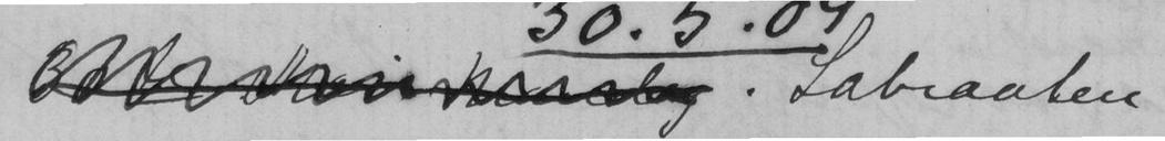. Labraaten
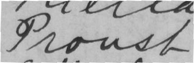Proust
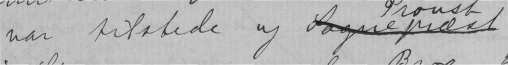var tilstede og
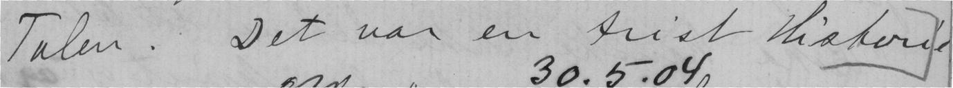Talen. Det var en trist Historie
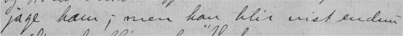jage ham; men han blir vist endnu
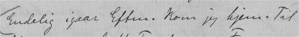Endelig igaar Eftm. kom jeg hjem. Til
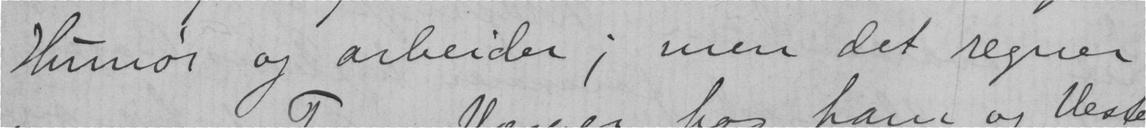Humør og arbeider; men det regner
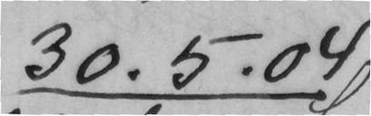30.5.04
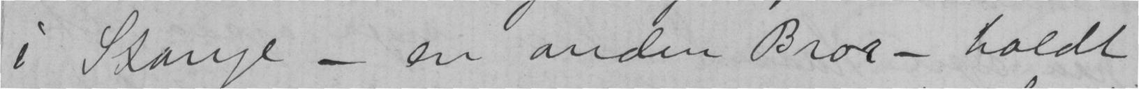i Stange - en anden Bror - holdt
